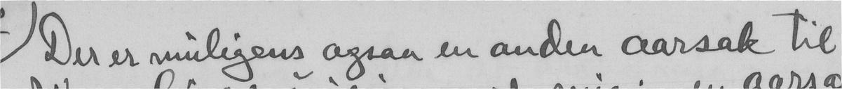Der er muligens ogsaa en anden aarsak til
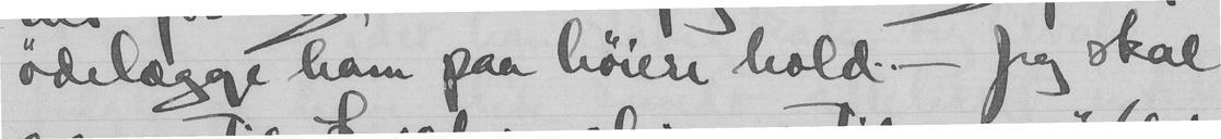ødelægge ham paa høiere hold. - Jeg skal
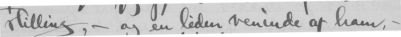stilling, - og en liden veninde af ham, -
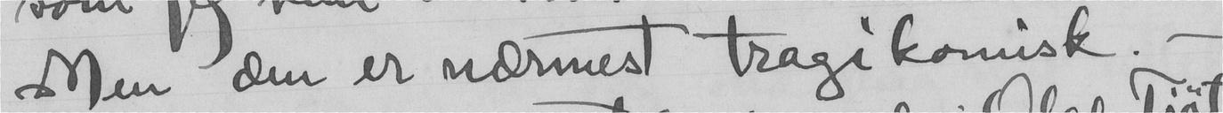Men den er nærmest tragikomisk. -
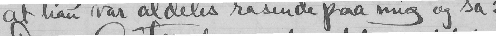at han var aldeles rasende paa mig og sa:
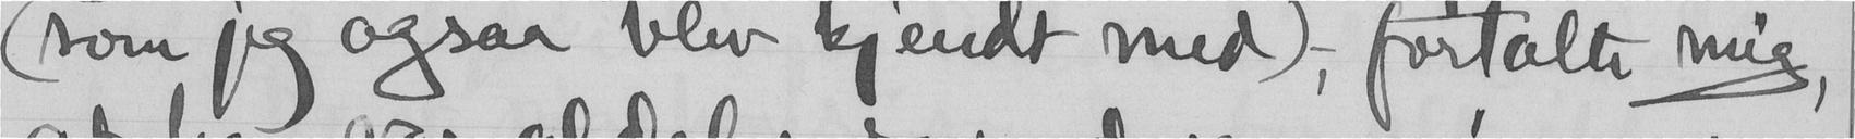(som jeg ogsaa blev kjendt med), fortalte mig,
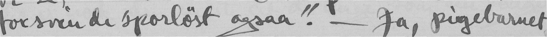forsvinde sporløst ogsaa." - Ja, pigebarnet
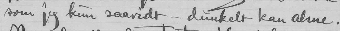som jeg kun saavidt - dunkelt kan alme.
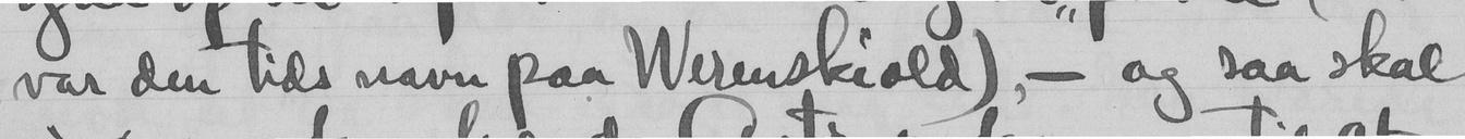var den tids navn paa Werenskiold), - og saa skal
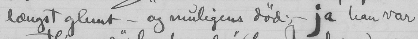længst glemt - og muligens død, - ja han var
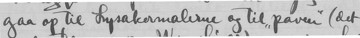gaa op til Lysakermalerne og til "paven" (det
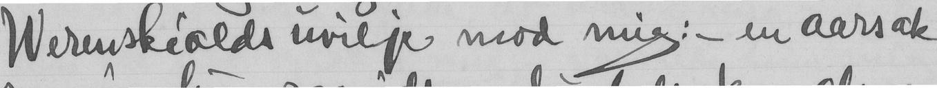Werenskiolds uvilje mod mig: - en aarsak
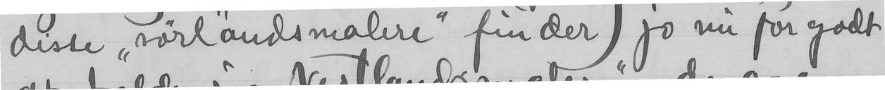disse "sørlandsmalere" finder jo nu for godt
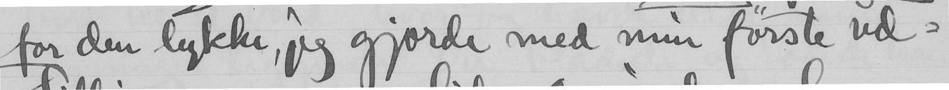for den lykke, jeg gjorde med min første ud-
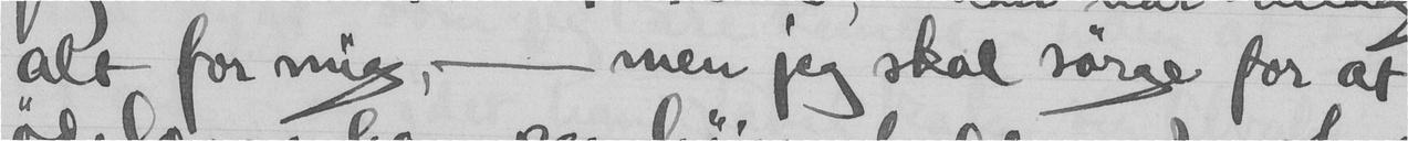alt for mig, - men jeg skal sørge for at
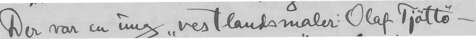Der var en ung "vestlandsmaler: Olaf Tjøttø -
